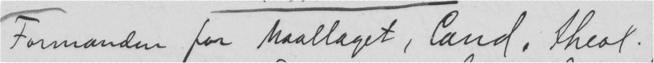Formanden for Maallaget, Cand. theol.
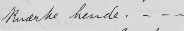kværke hende. — — —
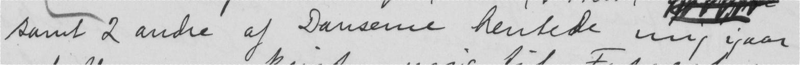samt 2 andre af Danserne hentede mig igaar
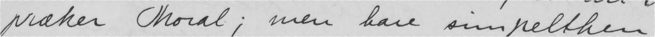præker Moral; men bare simpelthen
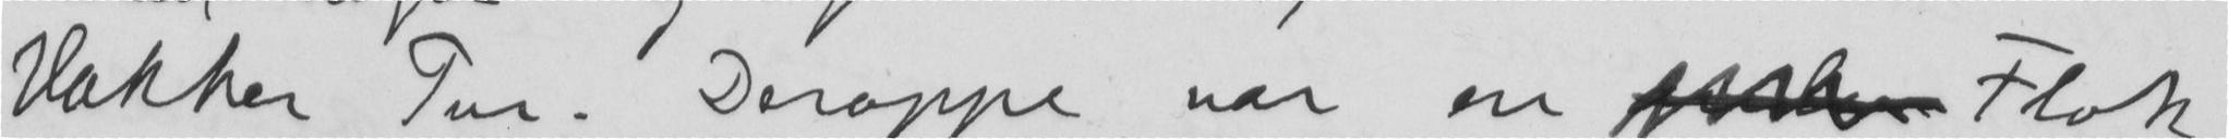Vakker Tun. Deroppe var en  Flok
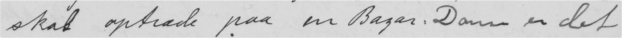skal optræde paa en Bazar. Dans er det
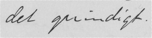det grundigt.
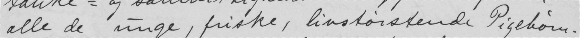alle de unge, friske, livstørstende Pigebørn.
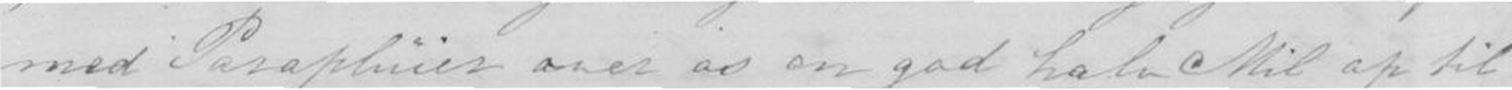med Parapluier over os en god halv Mil op til
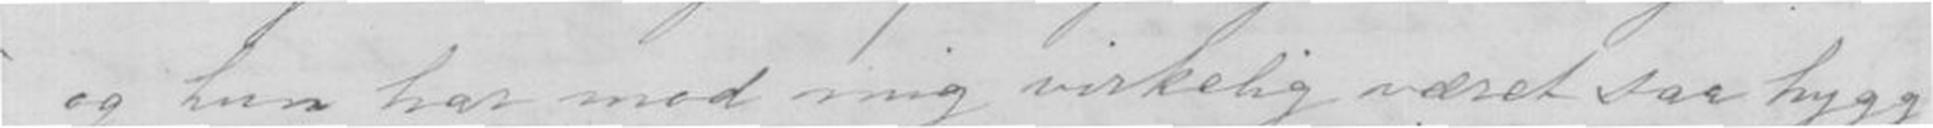og hun har mod mig virkelig været saa hygg-
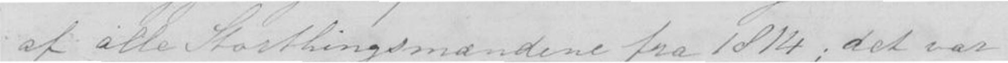af alle Storthingsmændene fra 1814; det var
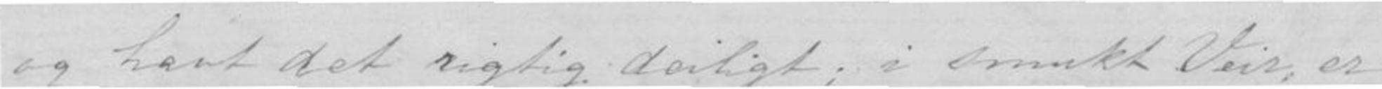og havt det rigtig deiligt; i smukt Veir, er
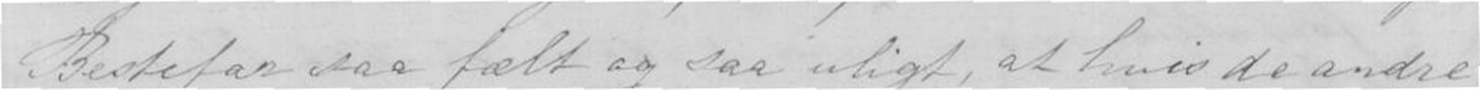Bestefar saa fælt og saa uligt, at hvis de andre
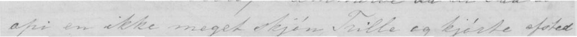opi en ikke meget Skjøn Trille og kjørte afsted
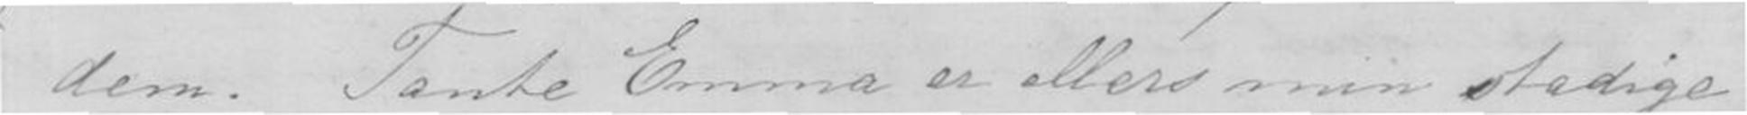dem. Tante Emma er ellers min stadige
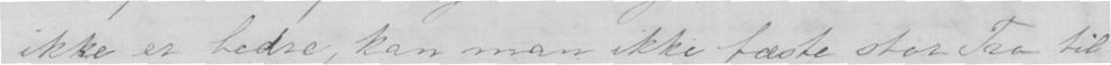ikke er bedre, kan man ikke fæste stor Tro til
